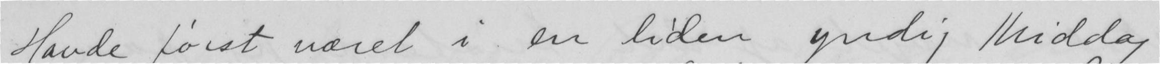Havde først været i en liden yndig Middag
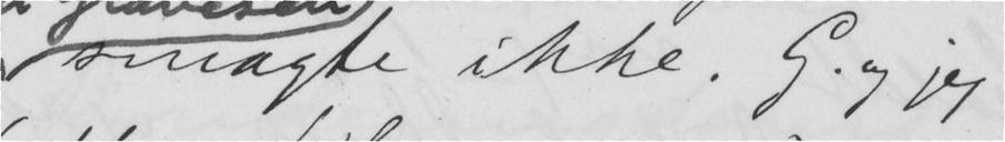smagte ikke. G. og jeg
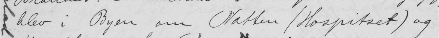blev i Byen om Natten (Hospitset) og
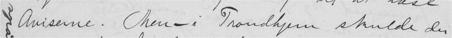Aviserne. Men - i Trondhjem skulde der
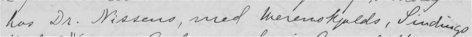hos Dr. Nissens, med Werenskjolds, Sindings
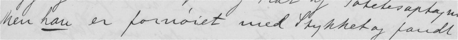Men han er fornøiet med Stykket og fandt
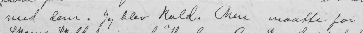med dem. Jeg blev kald. Men maatte for
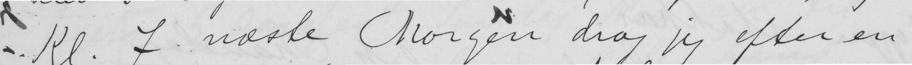Kl. 7 næste Morgen drog jeg efter en
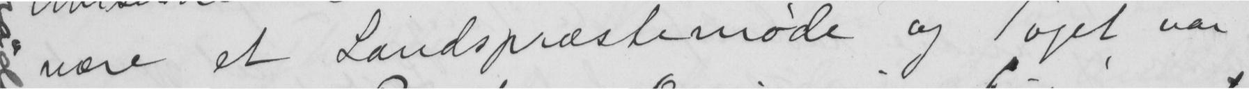være et Landspræstemøde og Toget var
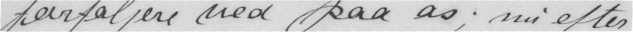farfoljere ned paa os; nu efter
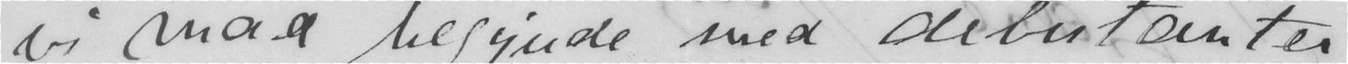vi maa begynde med debutanter,
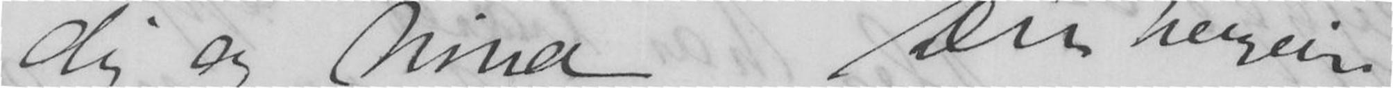dig og Nina Din hengivne
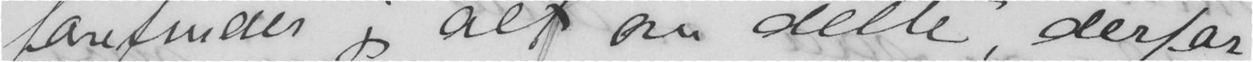forefinder jeg alt nu dette, derfor
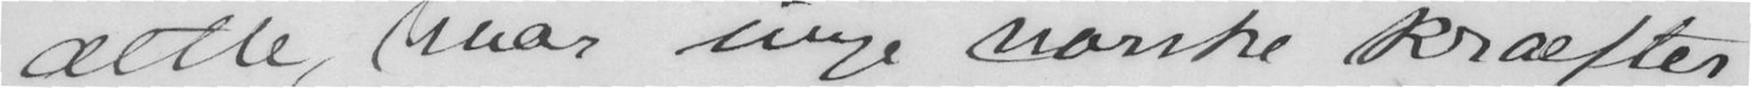dette, hvor unge norske kræfter
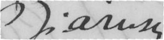Bj Bjørnson
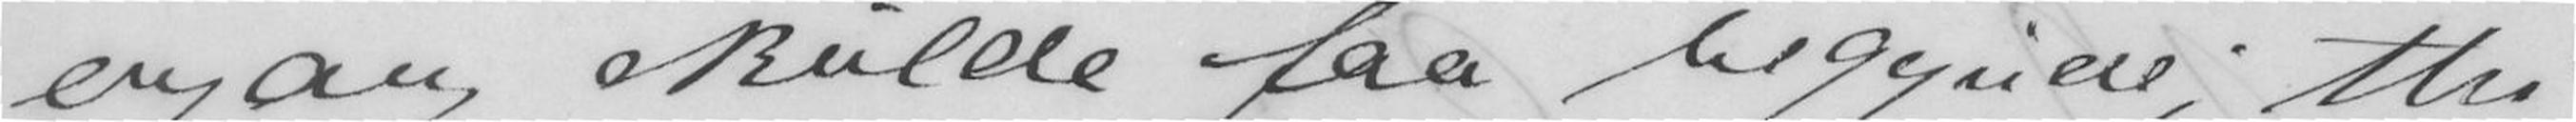engang skulde faa begynde, thi
